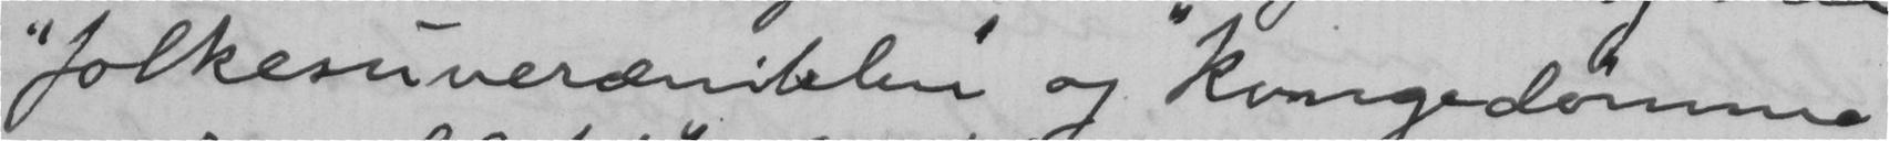"folkesuvereniteten" og "kongedømme
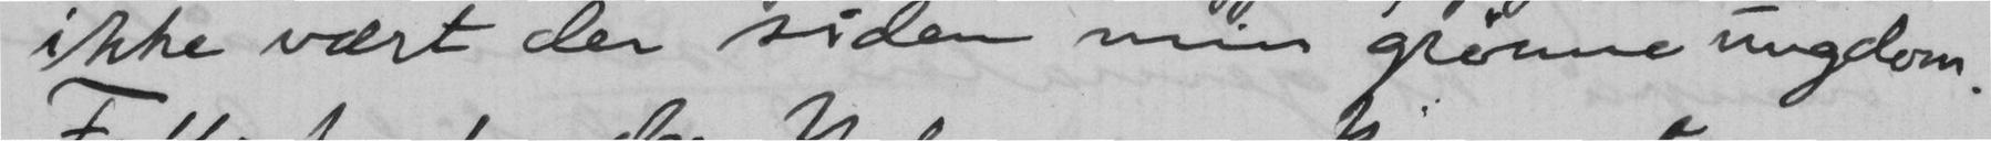ikke vært der siden min grønne ungdom.
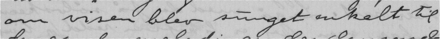om visen blev sunget enkelt til
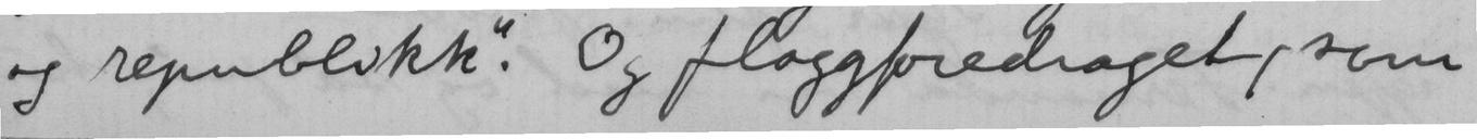og republikk". Og flaggforedraget, som
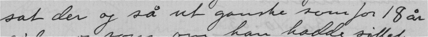sat der og så ut ganske som for 18 år
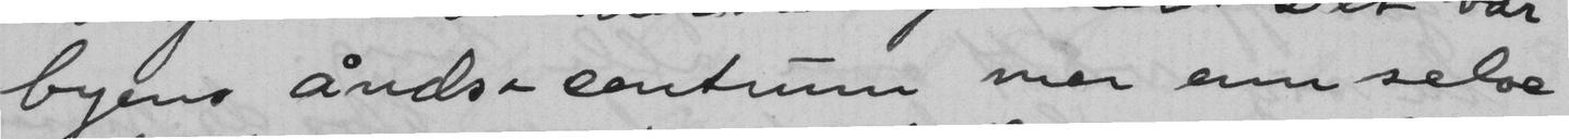byens ånds-centrum mer enn selve
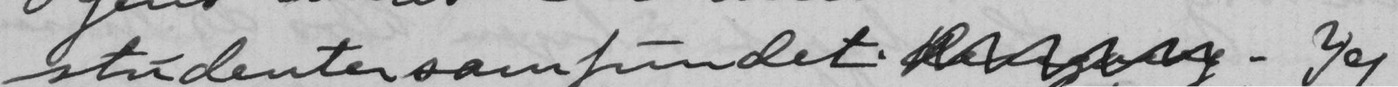studentersamfundet . Jeg
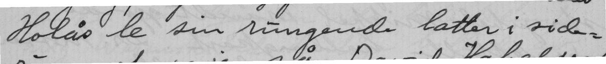Holås le sin rungende latter i side-
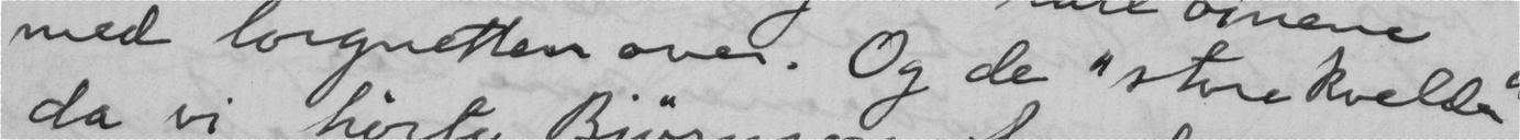med lorgnetten over. Og de "store kvelde"
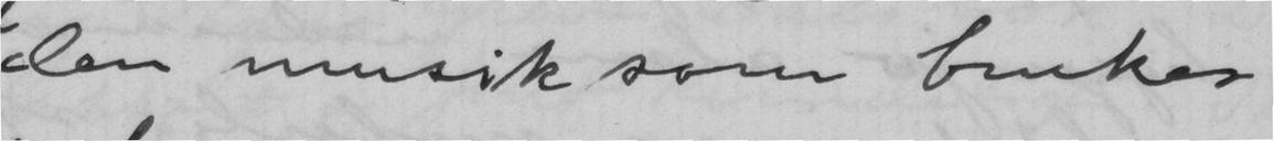den musik som brukes
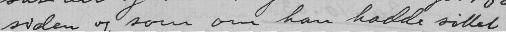siden og som om han hadde sittet
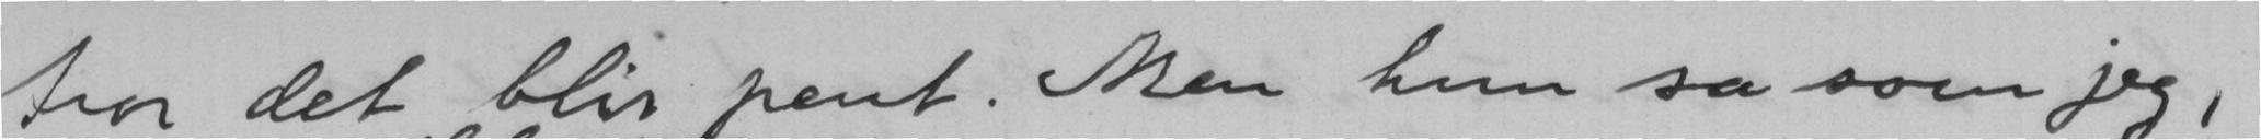tror det blir pent. Men hun sa som jeg,
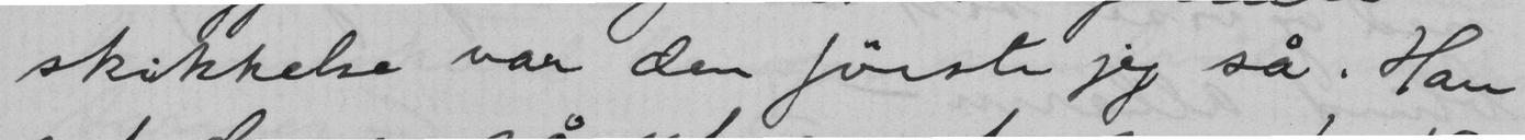skikkelse var den første jeg så. Han
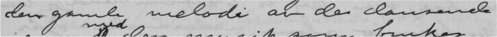den gamle melodi av de dansende
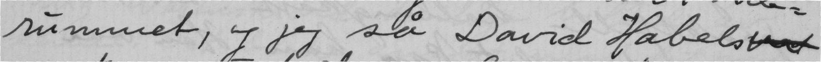rummet, og jeg så David Habels
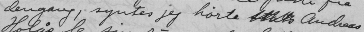dengang, syntes jeg hørde  Andreas
